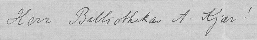Herr Bibliothekar A. Kjær!
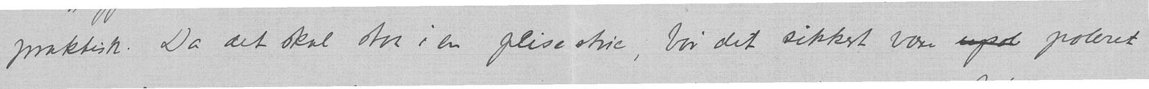praktisk. Da det skal staa i en peisestue, bør det sikkert være upol poleret
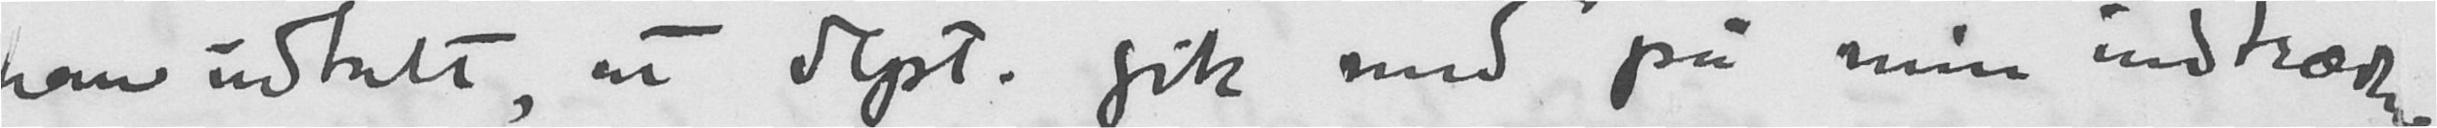han udtalt, at dept. gik med på min indtræden.
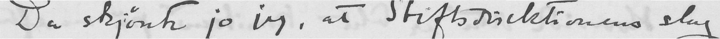Da skjønte jo jeg, at Stiftsdirektionens slag
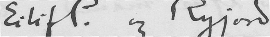Eilif P. og Ryjord
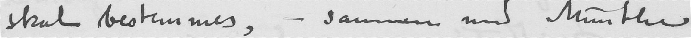skal bestemmes, - sammen med Munthe
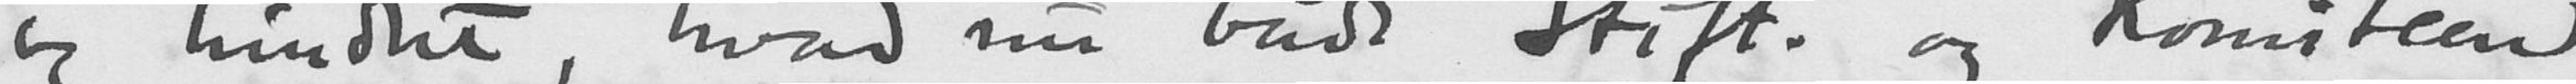og hindret, hvad nu både Stift. og Komiteen
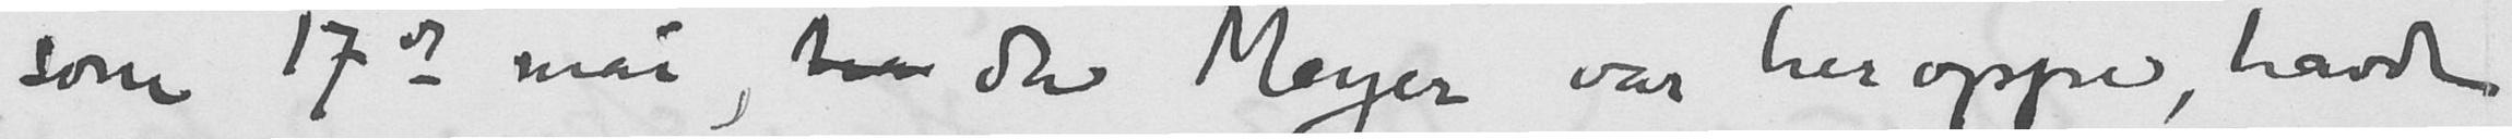som 17de mai, har da Meyer var her oppe, havde
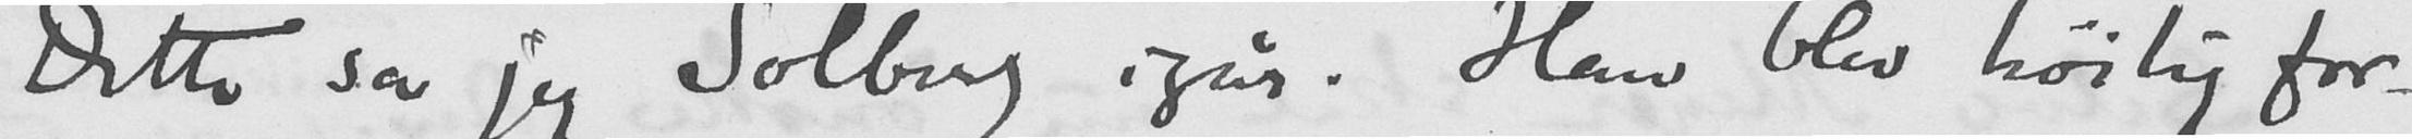Dette sa jeg Solberg igår. Han blev høilig for-
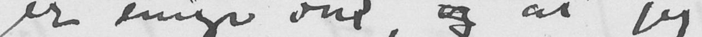er enige om, og at jeg
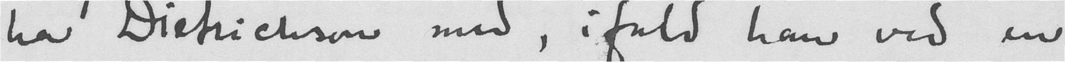ha Dietrichson med, ifald han ved en
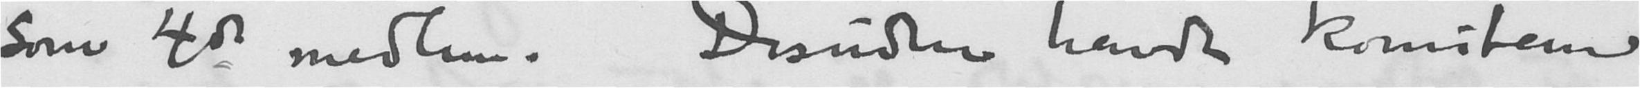Som 4de medlem. Desuden havde komiteen
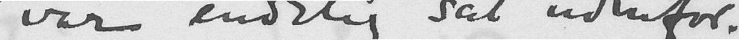var endlig sat udenfor.
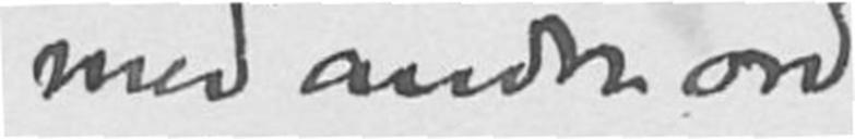med anre ord
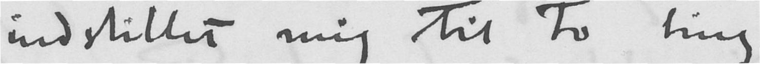indstillet mig til to ting.
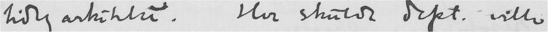tidig arkitekt. Hva skulde dept. ville
